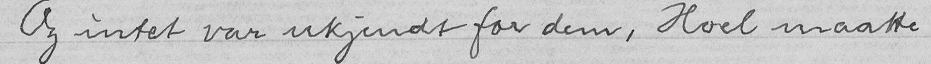Og intet var ukjendt for dem, Hoel maatte
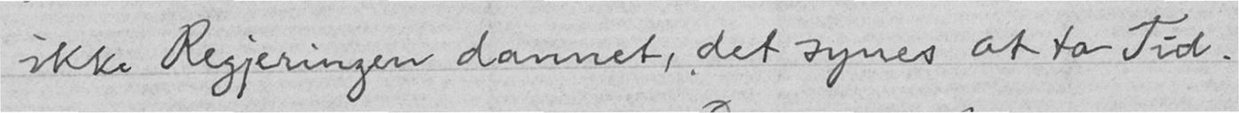ikke Regjeringen dannet, det synes at ta Tid.
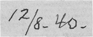12/8. 40.
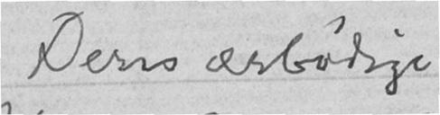Deres ærbødige
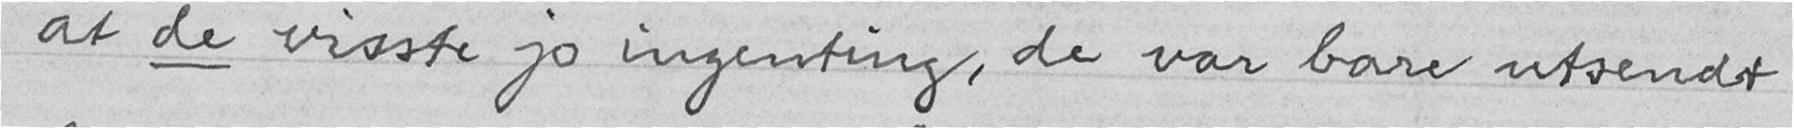at de visste jo ingenting, de var bare utsendt
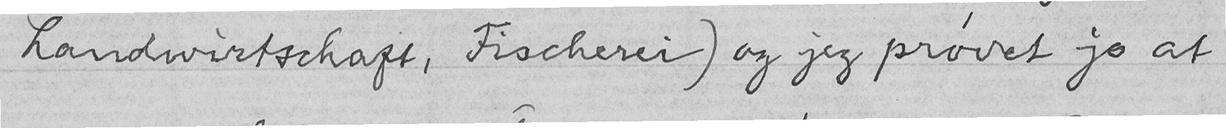Landwirtschaft, Fischerei) og jeg prøvet jo at
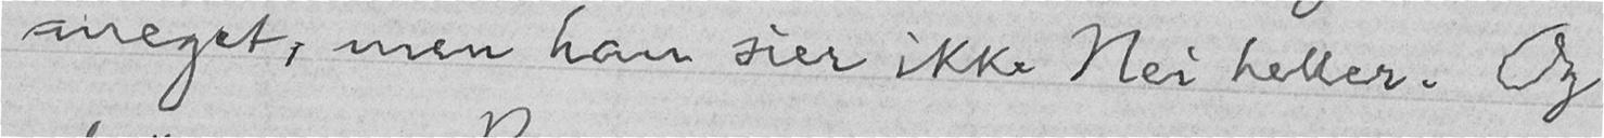meget, men han sier ikke Nei heller. Og
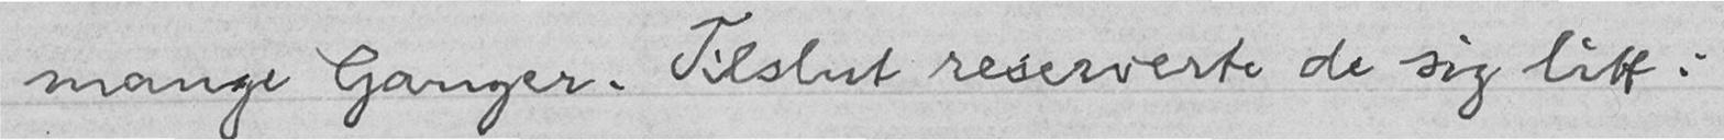mange Ganger. Tilslut reserverte de sig litt:
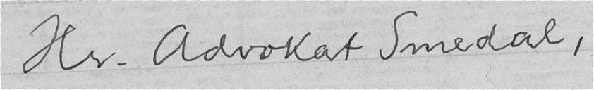Hr. Advokat Smedal,
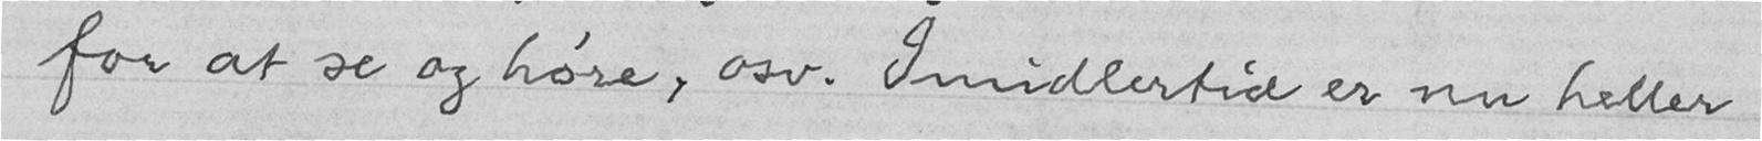for at se og høre, osv. Imidlertid er nu heller
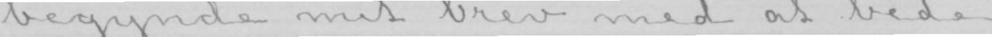begynde mit brev med at bede
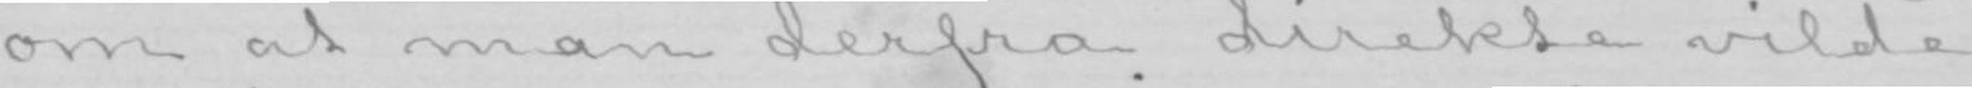om at man derfra direkte vilde
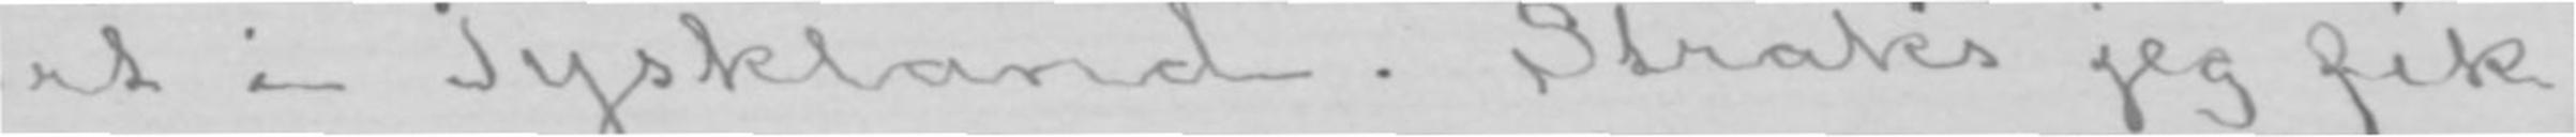gjort i Tyskland. Straks jeg fik
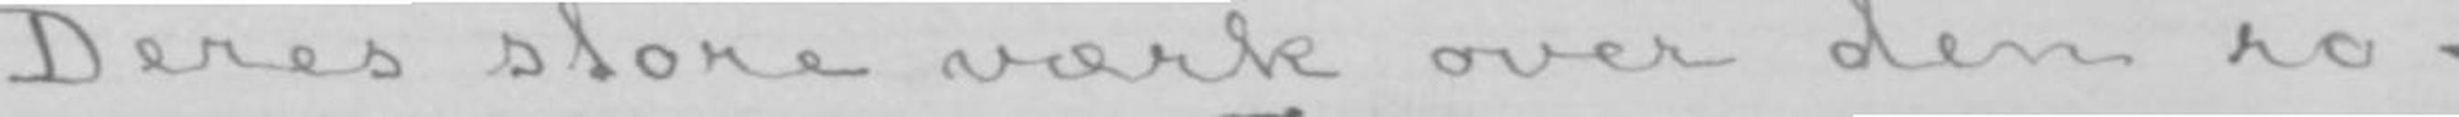Deres store værk over den ro-
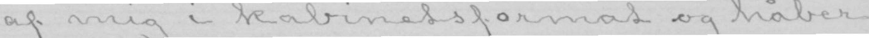af mig i kabinetsformat og håber
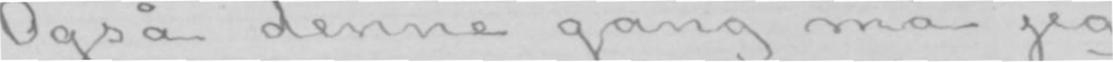Også denne gang må jeg
